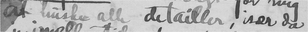at huske alle detailler, især da
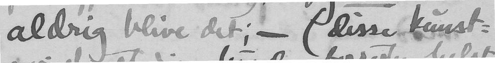aldrig blive det; - (disse kunst-
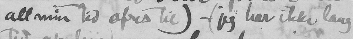all min tid ofres til) - (jeg har ikke lang
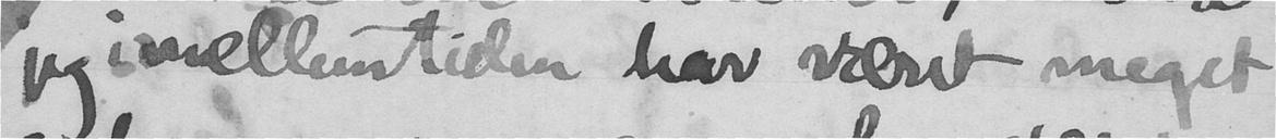jeg imellemtiden har været meget
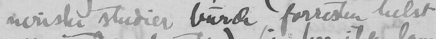neriske studier burde forresten helst
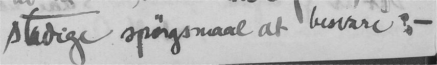stadige spørgsmaal at besvare: -
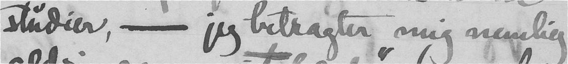studier, - jeg betragter mig nemlig
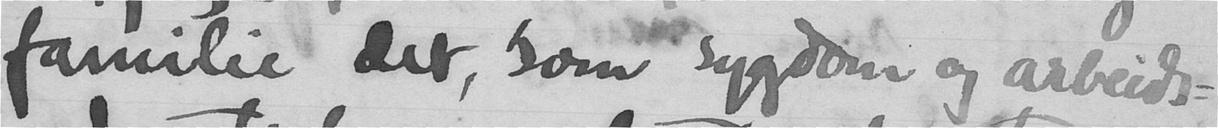familie det, som sygdom og arbeids-
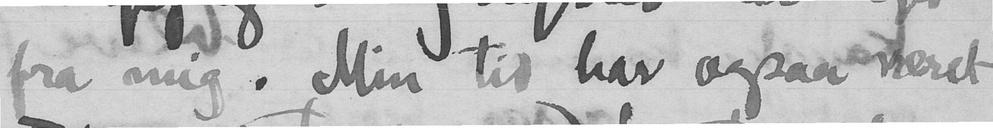fra mig. Min tid har ogsaa været
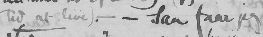tid at leve). - - Saa faar jeg
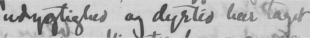udygtighed og dyrtid har taget
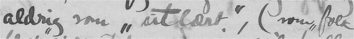aldrig som "utlært", (som "folk
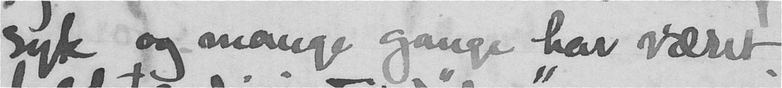syk og mange gange har været
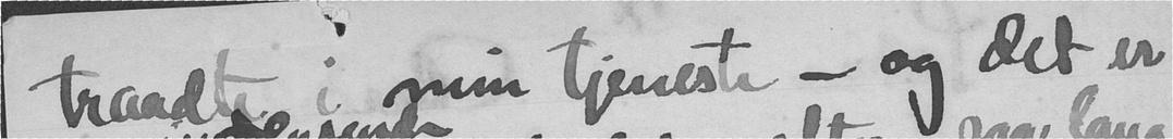traadte i min tjeneste - og det er
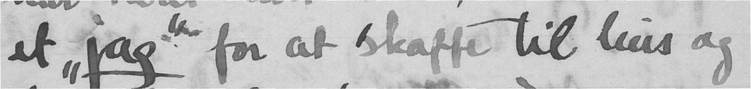et "jag" for at skaffe til hus og
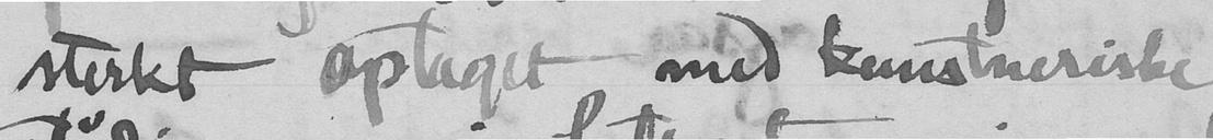sterkt optaget med kunstneriske
